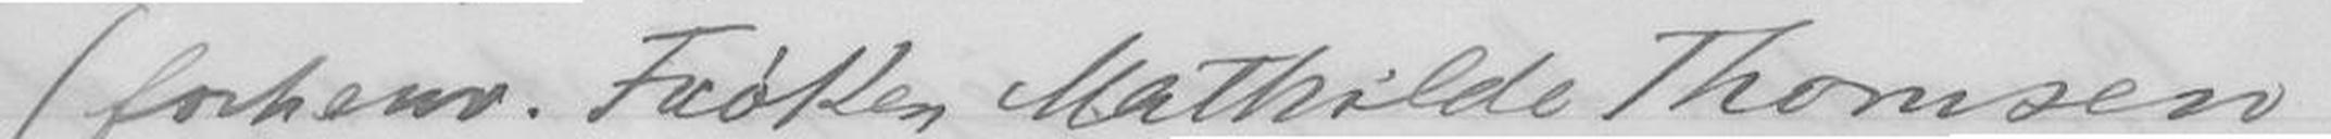(forhenv. Frøken Mathilde Thomsen
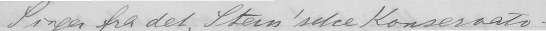Siger fra det "Stein'ske Konservato-
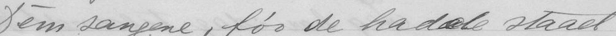Dem sangene, før de hadde staaet
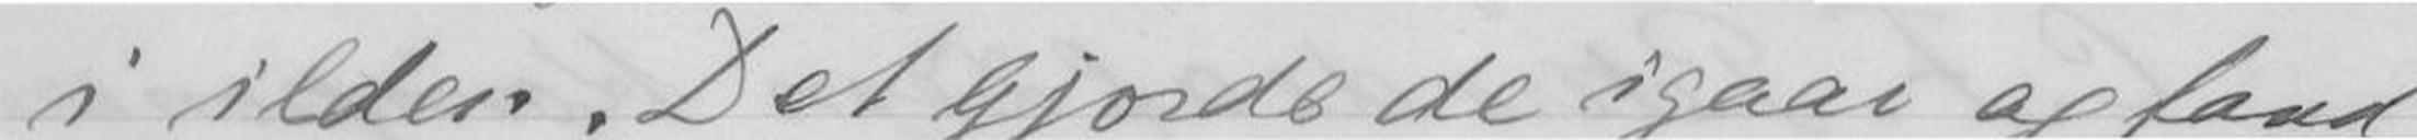i ilden. Det gjorde de igaar og fand
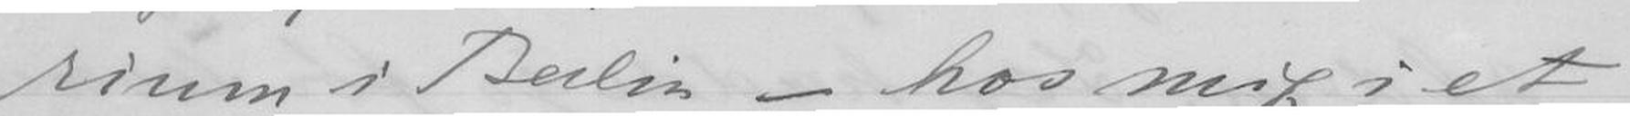rium i Berlin - hos mig i et
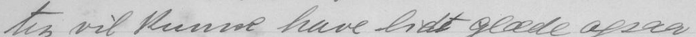ten vil kunne have lidt glæde ogsaa
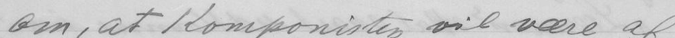om, at Komponister vil være af
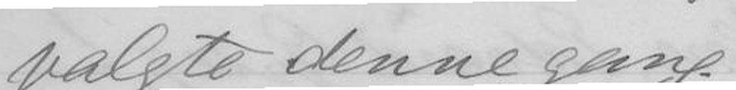valgte denne gang.
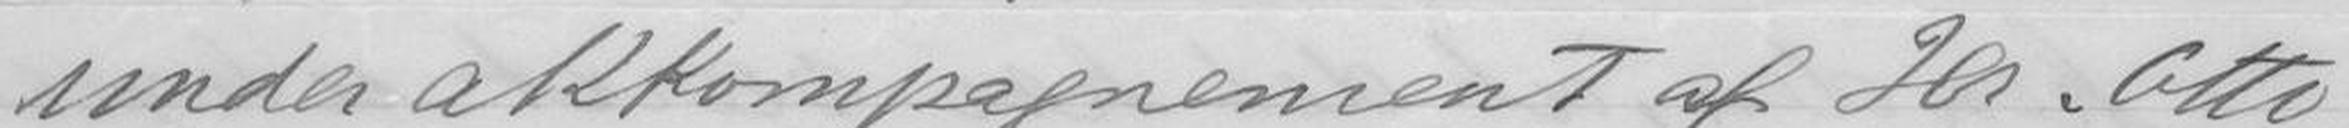under akkompagnement af hr. Otto
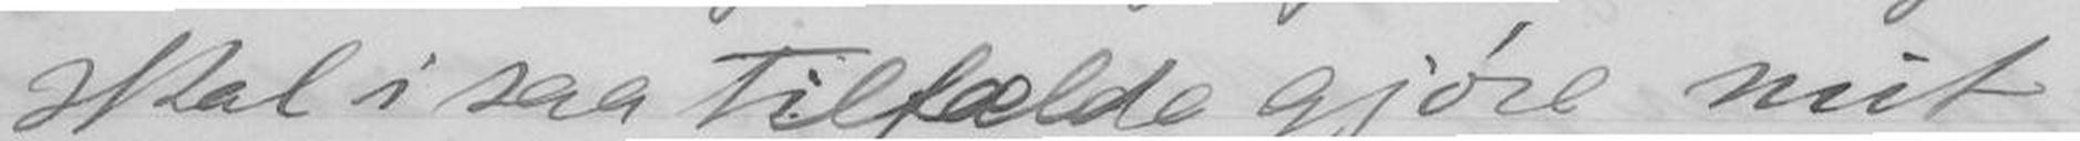skal i saa Tilfælde gjøre mit
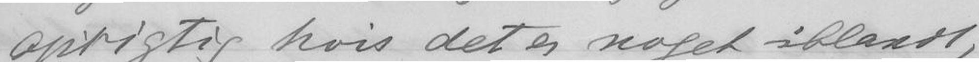oprigtig, hvis det er noget iblandt,
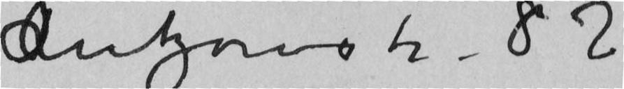Lutzowstr. 82
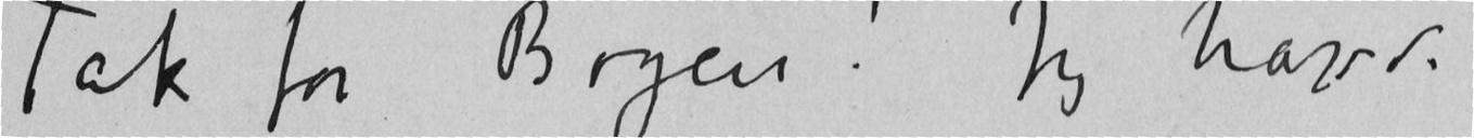Tak for Bogen! Jeg havde
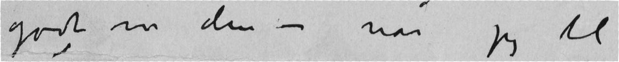godt om den – når jeg til
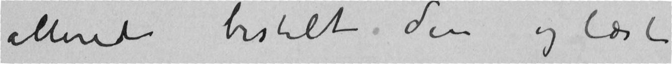allerede bestilt den og læst
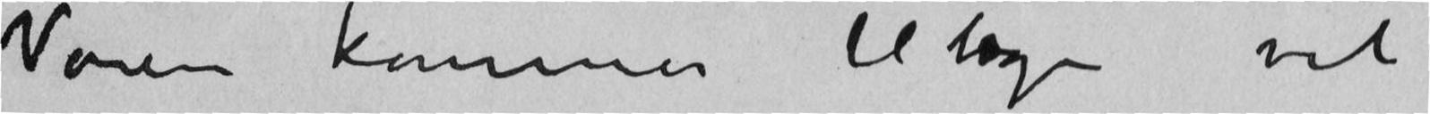Våren kommer tilbake vil
